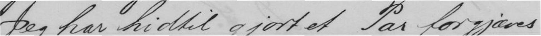Jeg har hidtil gjort et Par forgjæves
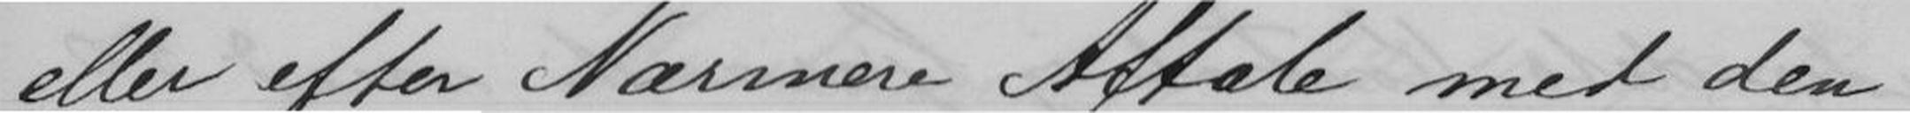eller efter Nærmere Aftale med den
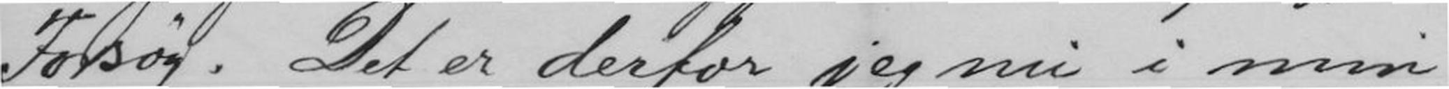Forsøg. Det er derfor jeg nu i min
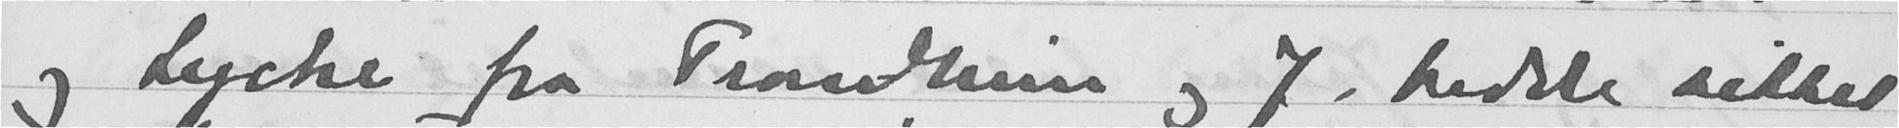og Lyche fra Tronidhim og J. hadde sittet
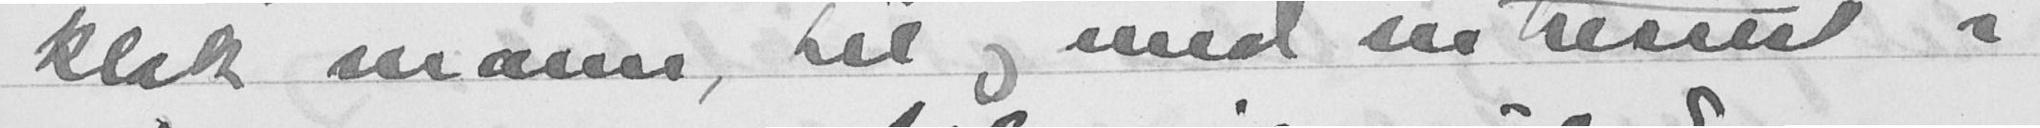klok mann, til og med intressert i
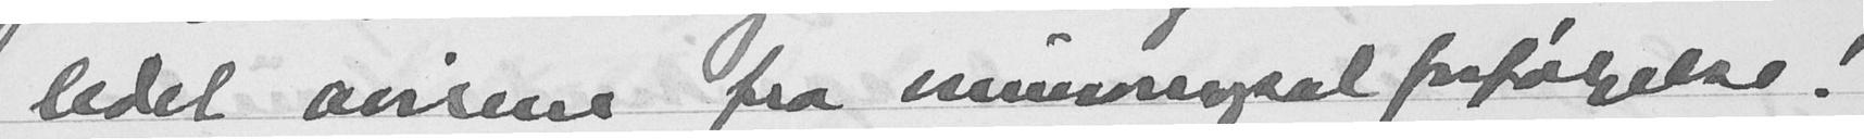ledet avisene fra minoritetsforfølgelse!
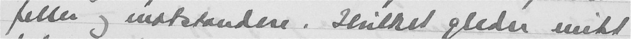feller og motstandere. Hvilket gleder mitt
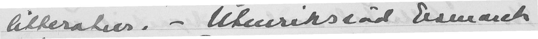litteraturen. - Utenriksråd Esmarck
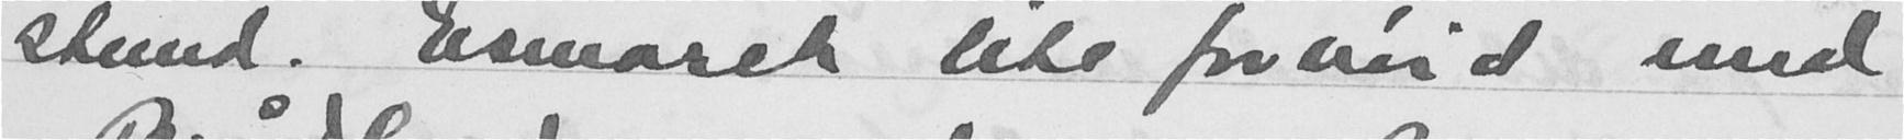stund. Esmarck lite fornøid med
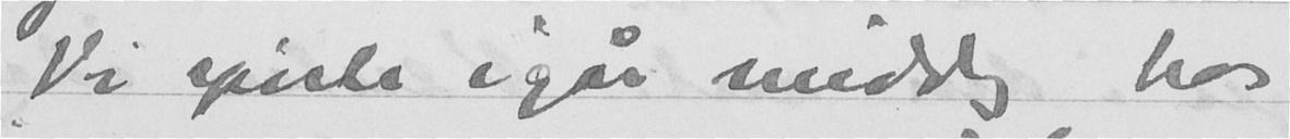Vi spiste igår middag hos
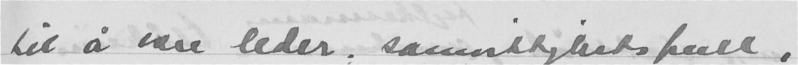til å være leder, samvittighetsfull,
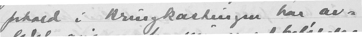forhold i Kringkastingen har av-
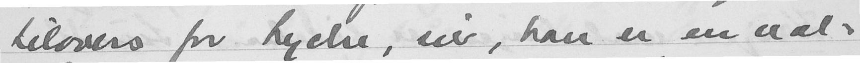tilovers for Lyche, sier, han er en ual-
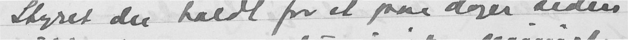Styret der holdt for et par dager siden
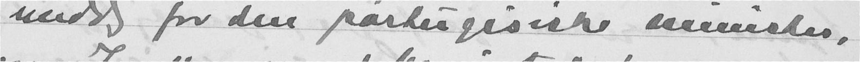middag for den portugisiske minister,
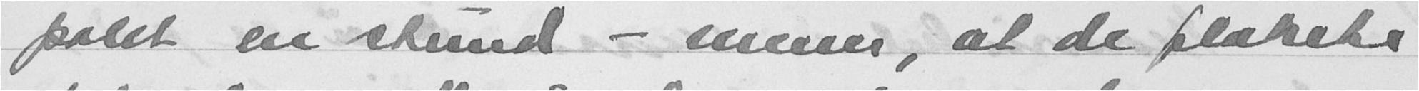polet en stund - mener, at de flokete
